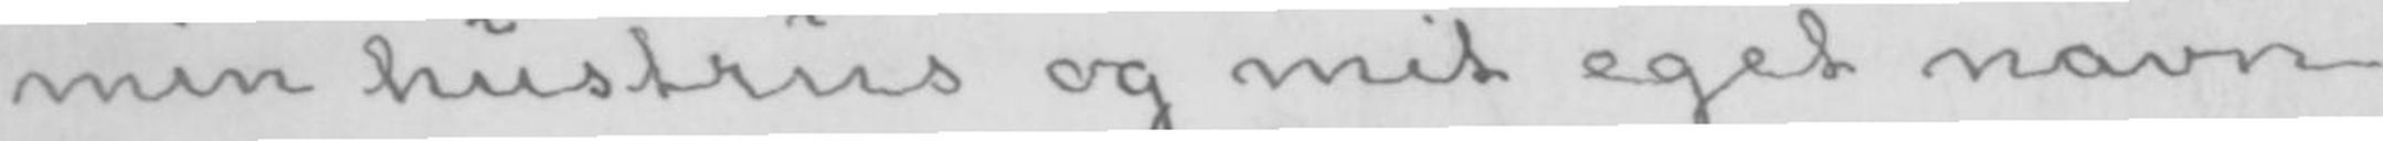min hustrus og mit eget navn
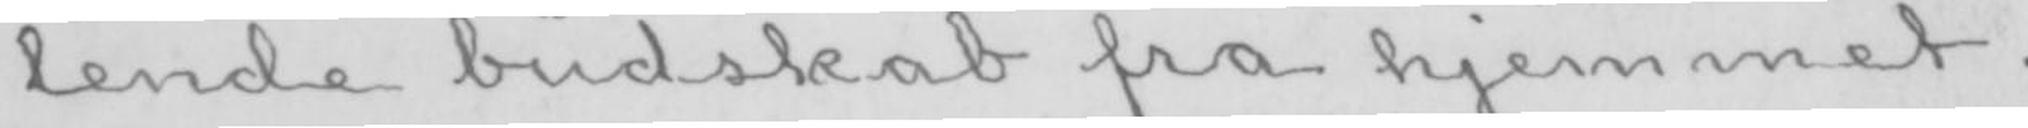lende budskab fra hjemmet.
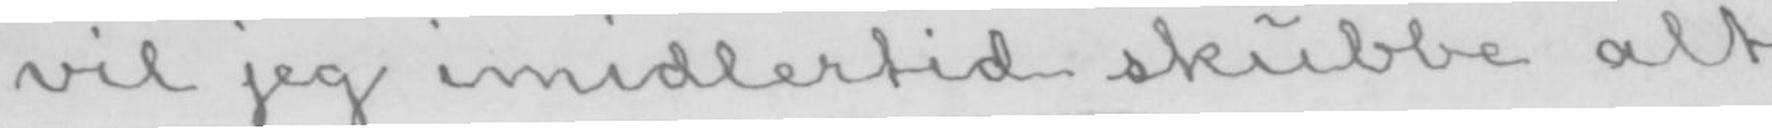vil jeg imidlertid skubbe alt
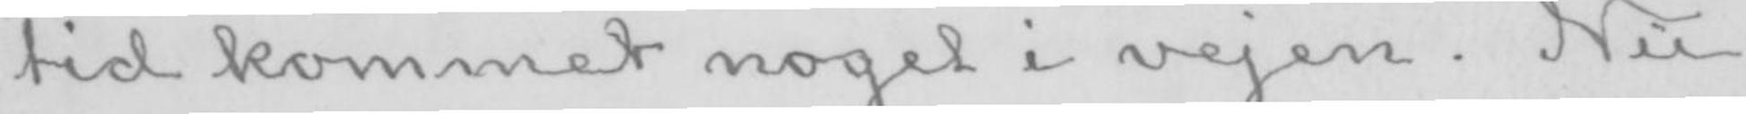tid kommet noget i vejen. Nu
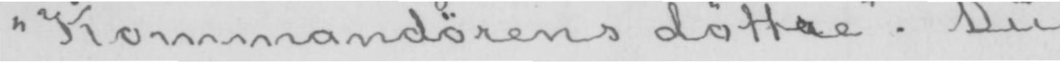«Kommandørens døttere». Du
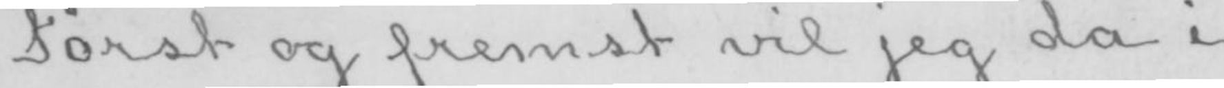Først og fremst vil jeg da i
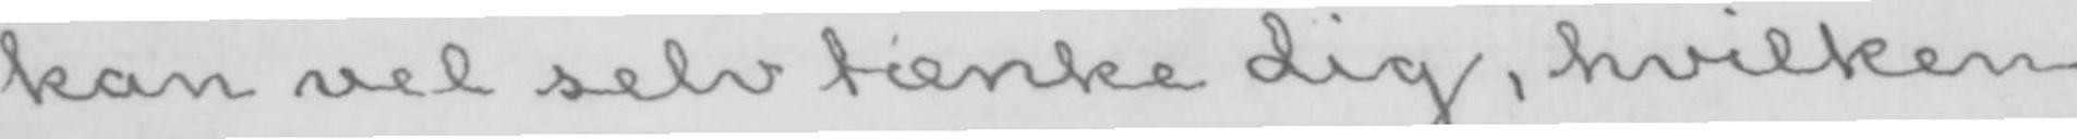kan vel selv tænke dig, hvilken
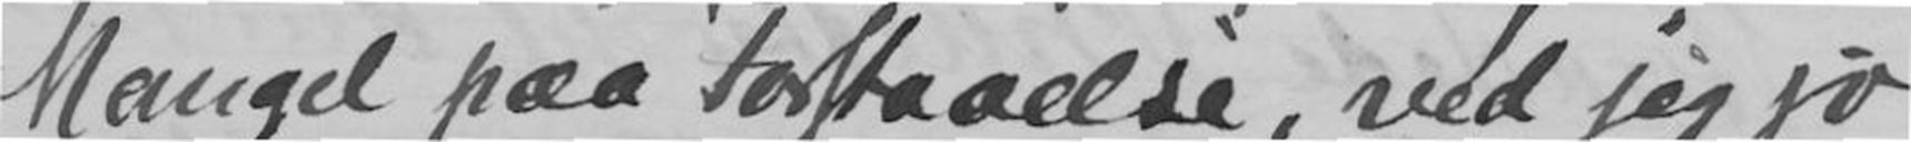Mangel paa Forstaaelse, ved jeg jo
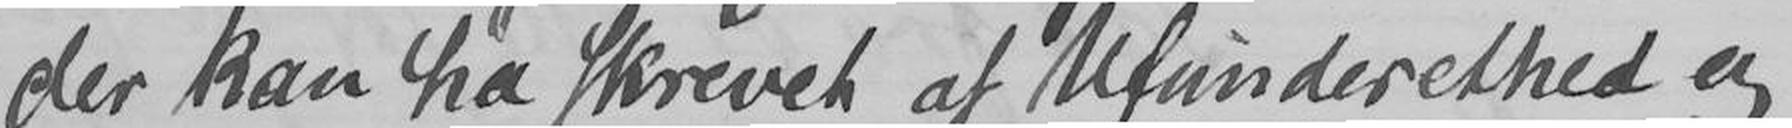der kan ha skrevet af Ufunderethed og
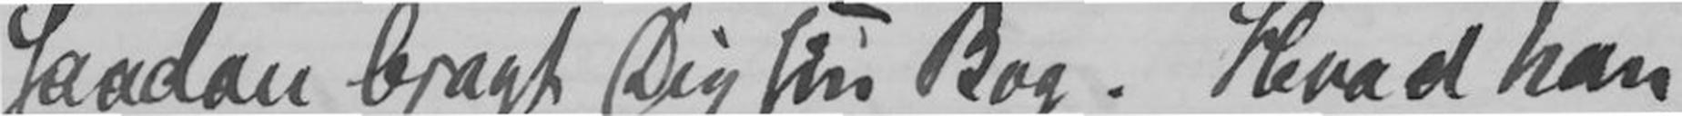saadan bragt Dig sin Bog. Hvad han
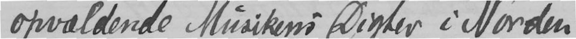opvældende Musikens Digter i Norden
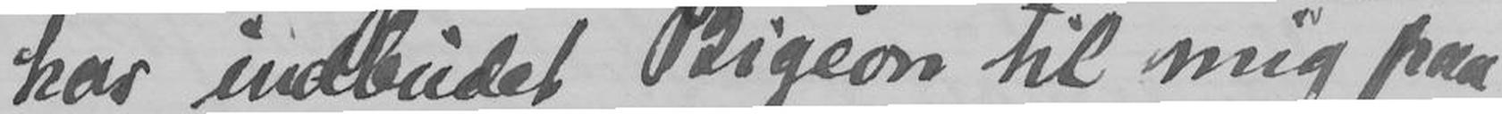har indbudet Bigeon til mig paa
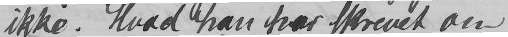ikke. Hvad han har skrevet om
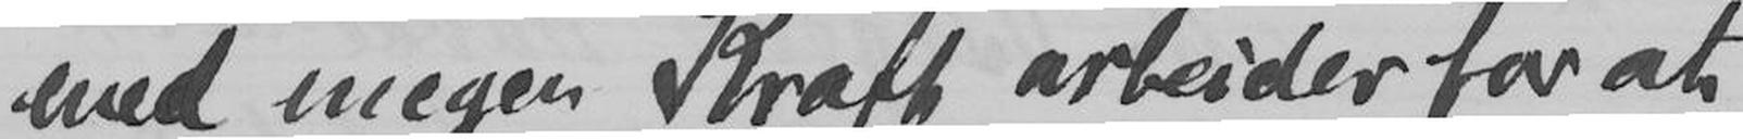med megen Kraft arbeider for at
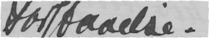Forstaaelse.
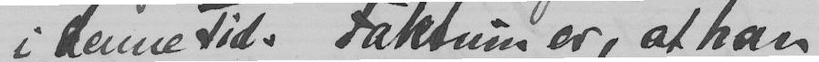i denne Tid. Faktum er, at han
